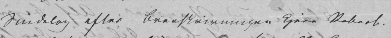Sindelag efter Brevskrivningen kjere Robert.
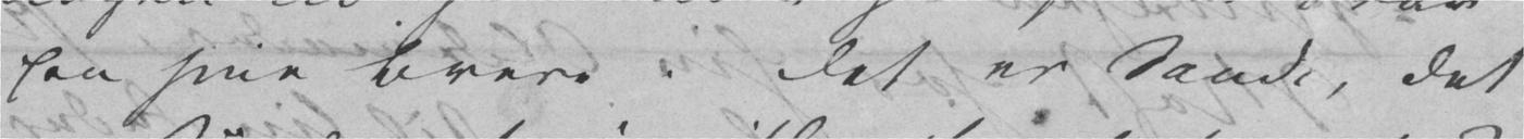paa sine Breve. Det er Sandt, det
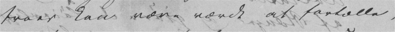troer kan være værdt at fortælle,
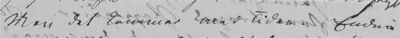Men det kommer med Tiden. Endnu
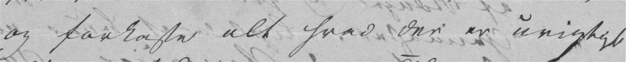og forkaste alt hvad der er uvigtigt.
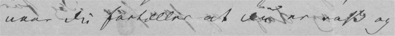naar Du fortæller at Du er rask og
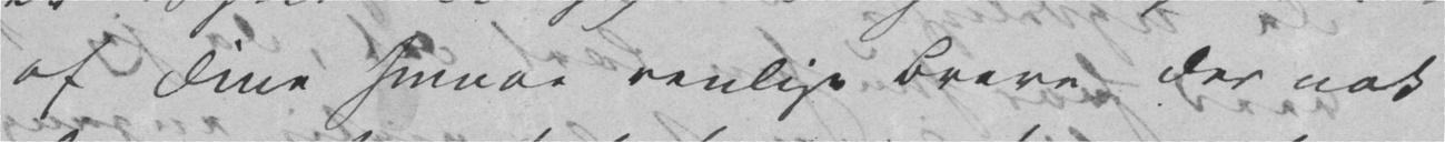af dine smaae venlige Breve der nok
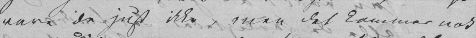vare de just ikke, men det kommer nok
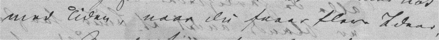med Tiden, naar du faaer flere Ideer,
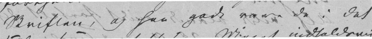Skriften, og saa godt vare de i det
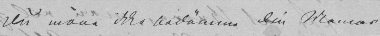Du maae ikke bedømme din Mamas
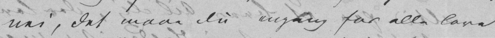nei, det maae Du engang for alle love
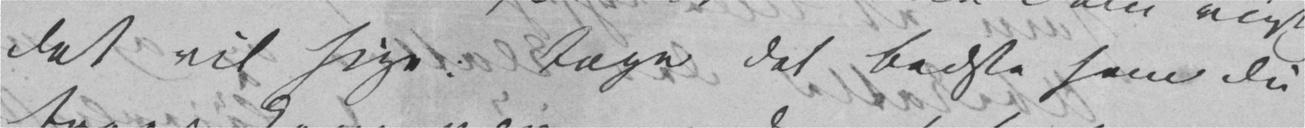det vil sige: tage det bedste som Du
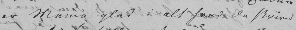er Mama glad i alt hvad Du skriver
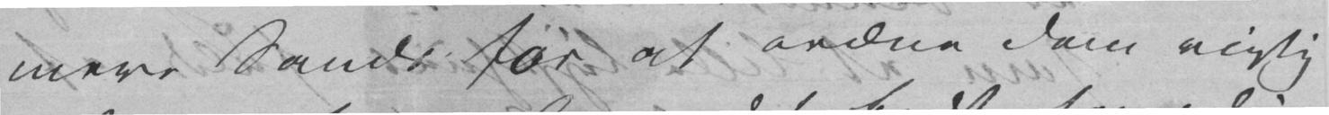mere Sands for at ordne dem rigtig
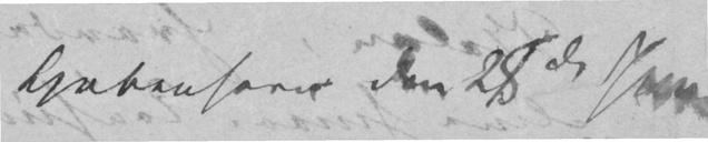Kjøbenhavn den 28de Juni
